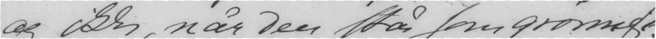og ikke, når den står som grønnest.
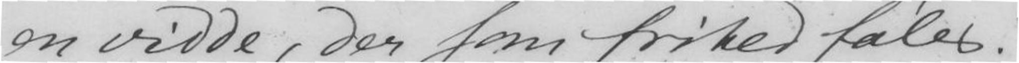en vidde, der som frihed føles!
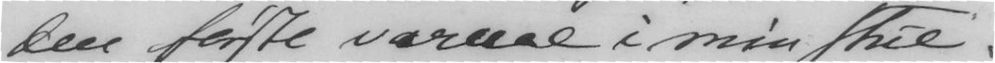den første varme i min stue.
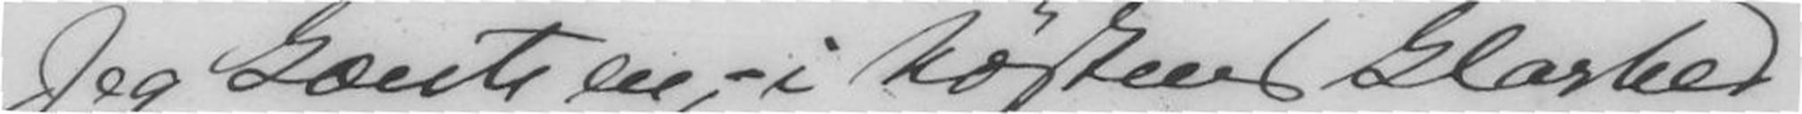Jeg kænte en, - i høstens klarhed
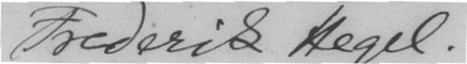Frederik Hegel.
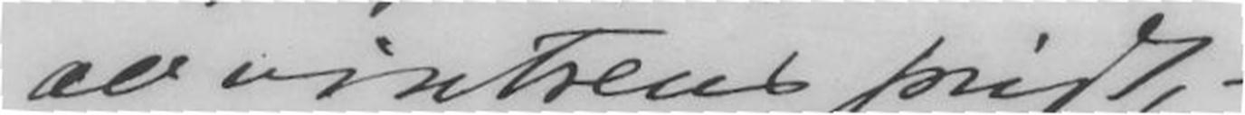av vintrens pust, -
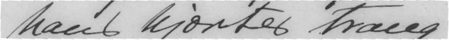hans hjærtes trang
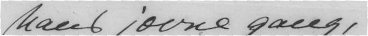hans jævne gang,
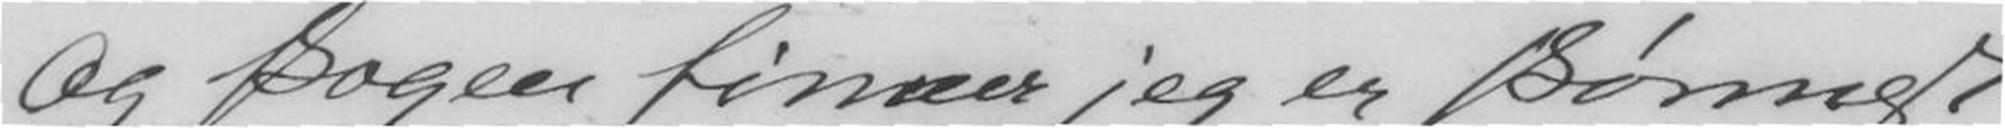Og skogen finner jeg er skønnest
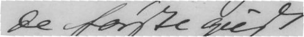de første gust
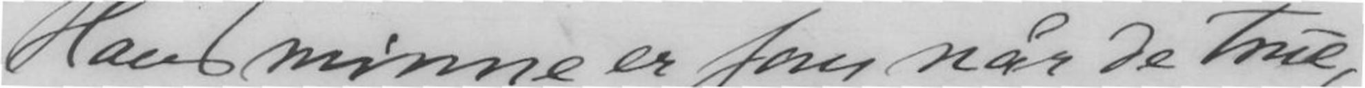Hans minne er som når de true,
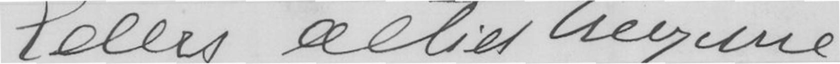Eders altid hengivne
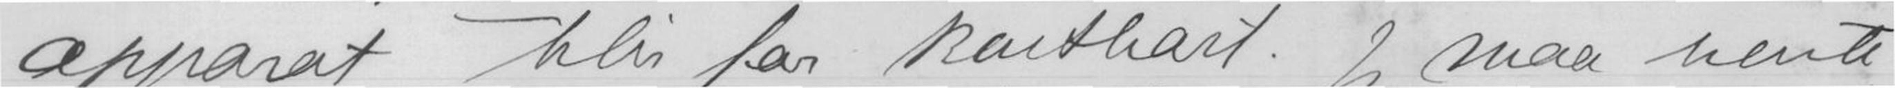apparat blir for kostbart. Jeg maa vente
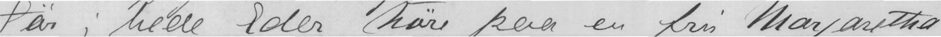Tør jeg bede Eder høre paa en fru Margaretha
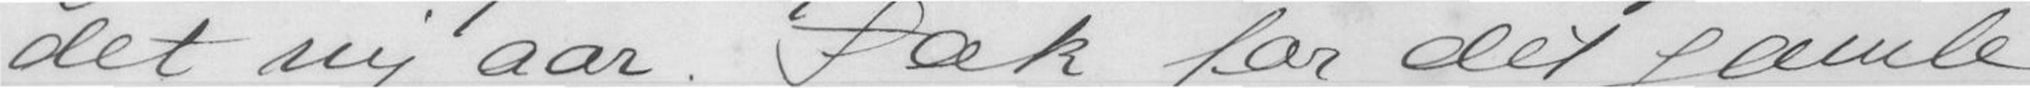det ny aar. Tak for det gamle.
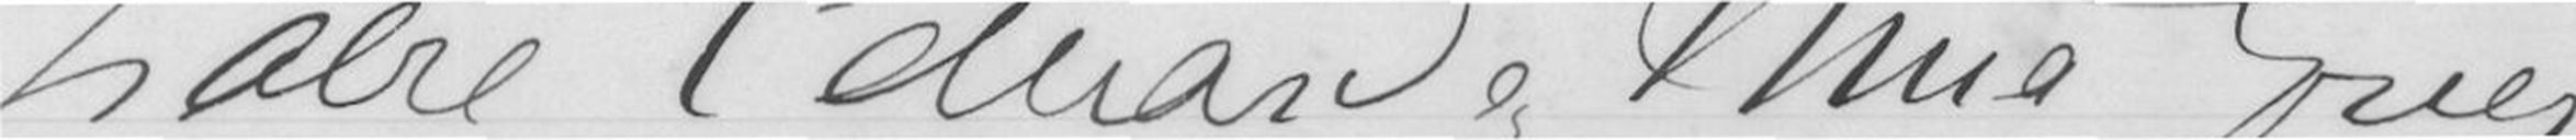Kjære Edvard og Nina Grieg
